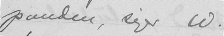panden, siger W.
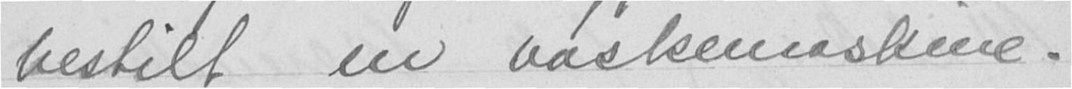bestilt en vaskemaskine.
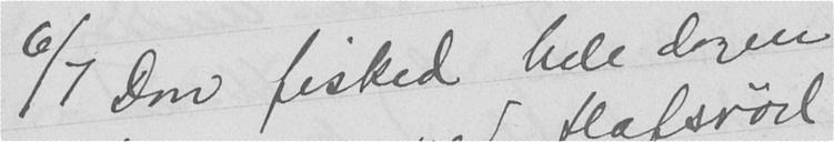6/7 Don fisked hele dagen
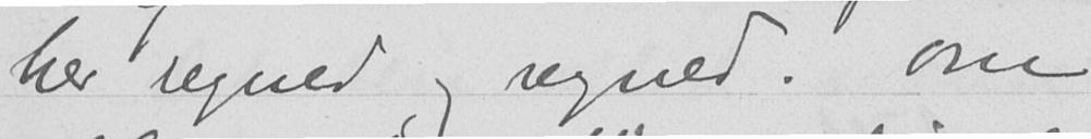her regned og regned. Om
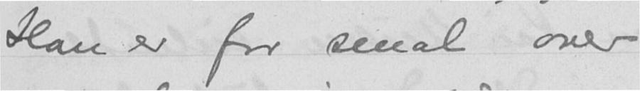Han er for smal over
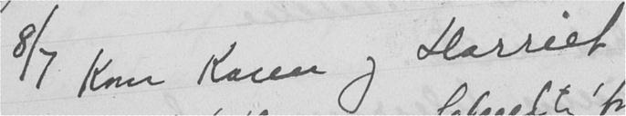8/7 Kom Karen og Harriet
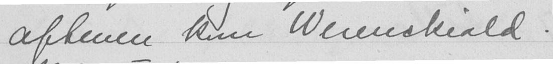aftenen kom Werenskiold.
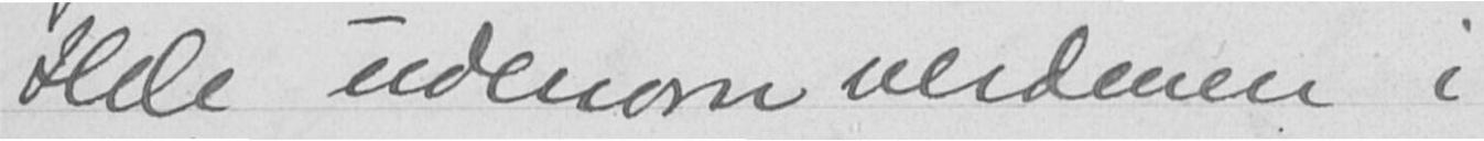Hele udenomverdenen i
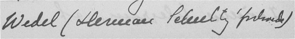Wedel (Herman Schultz' forlovede)
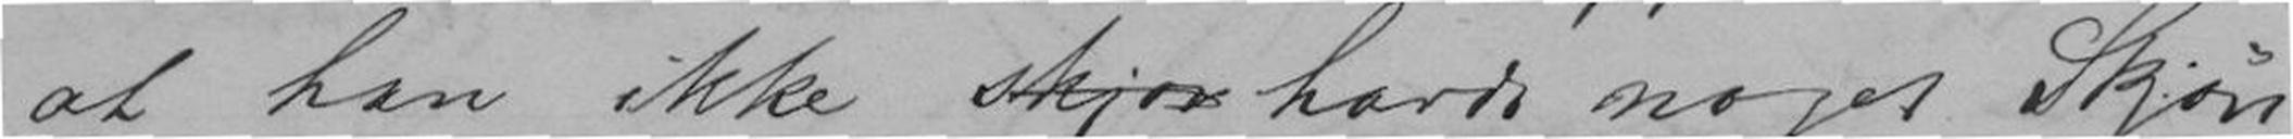at han ikke skjøn havde noget Skjøn
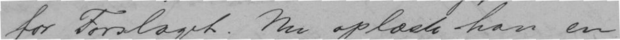for Forslaget. Nu oplæste han en
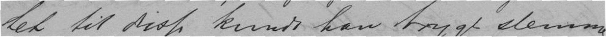tet til disse kunde han trygt stemme
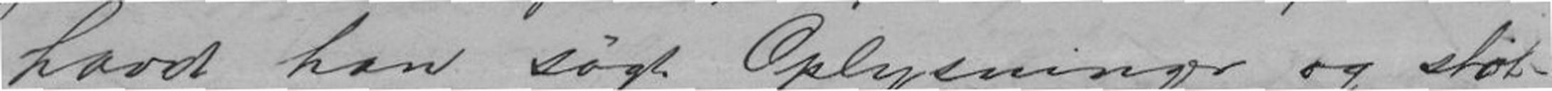havde han søgt Oplysninger og støt-
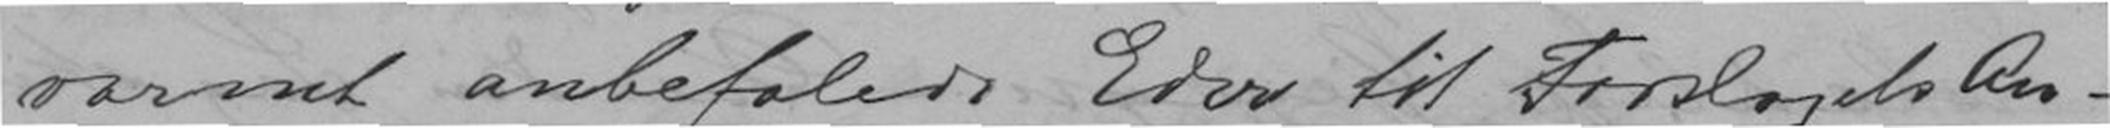varmt anbefalede Eder til Forslagets An-
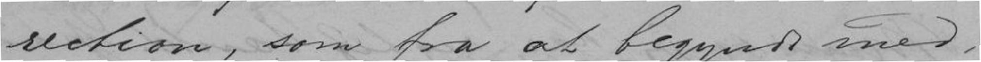rection, som fra at begynde med,
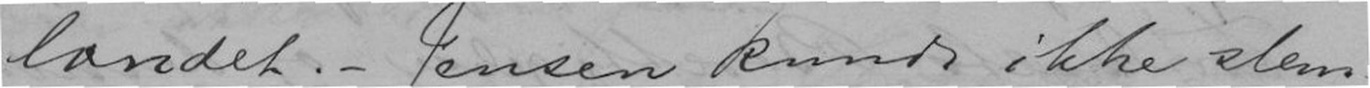landet. - Jensen kunde ikke stem
